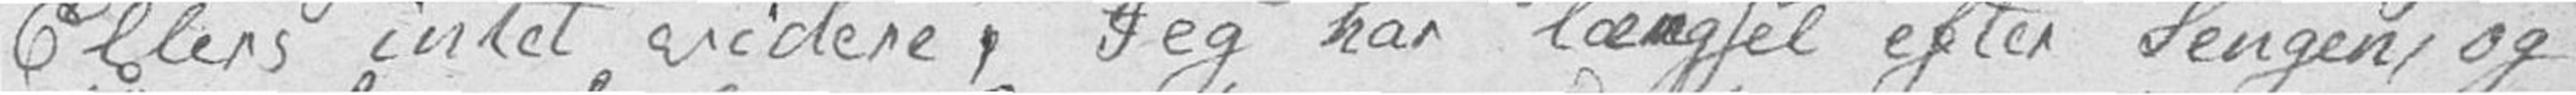Ellers intet videre, Jeg har længsel efter Sengen, og
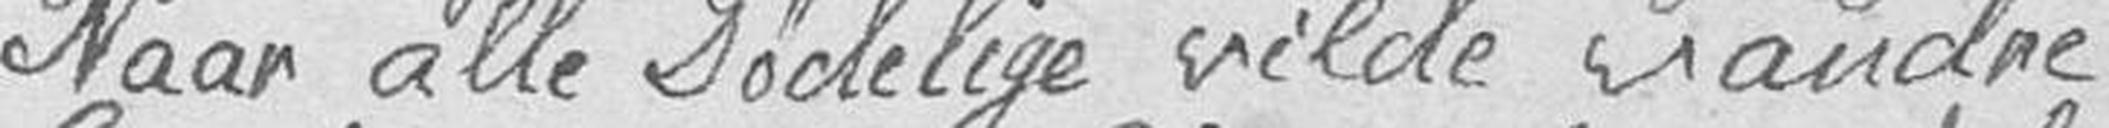Naar alle Dødelige vilde vandre
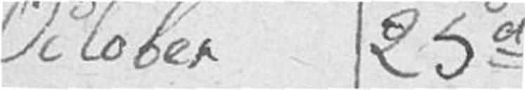October 25d
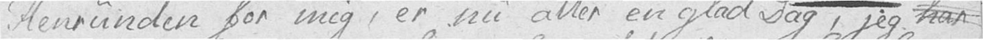Henrunden for mig, er nu atter en glad Dag, jeg har
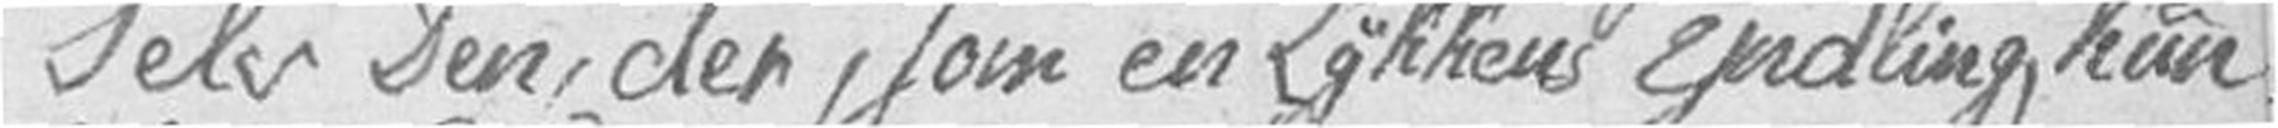Selv Den, der, som en Lykkens yndling, kun
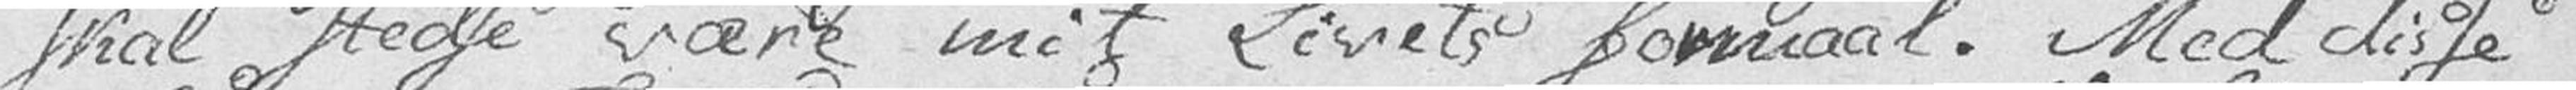skal stedse være mit Livets formaal. Med disse
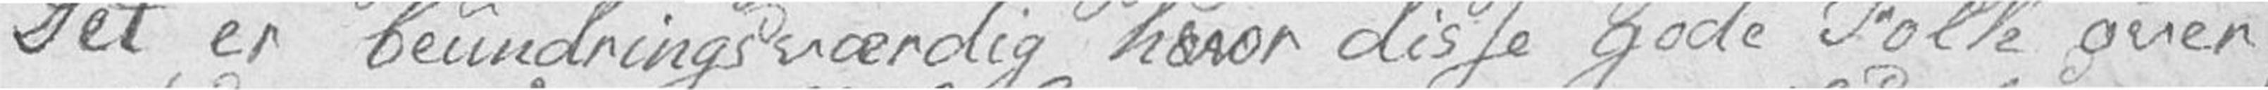Det er beundringsværdig hvor disse gode Folk over
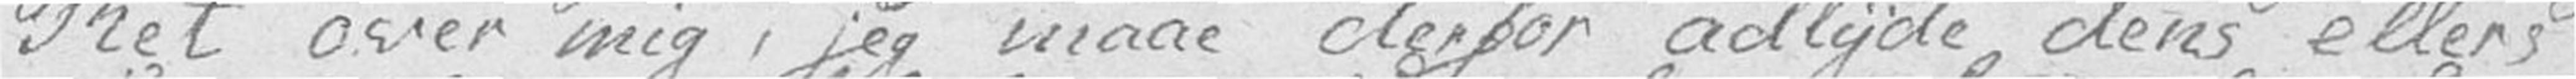Ret over mig, jeg maae derfor adlyde dens ellers
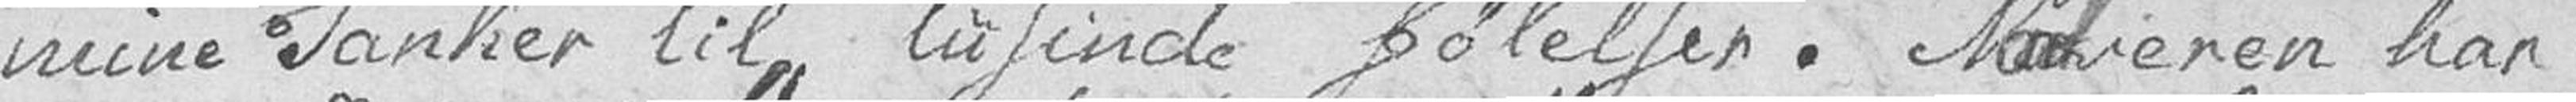mine Tanker til tusinde følelser. Nadveren har
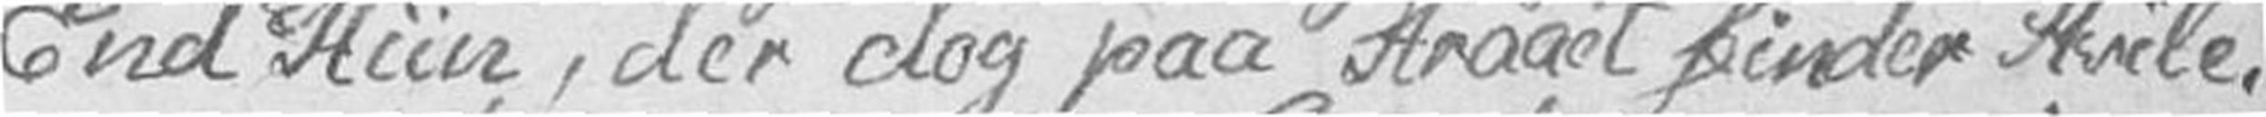End Hiin, der dog paa Straaet finder Hvile.
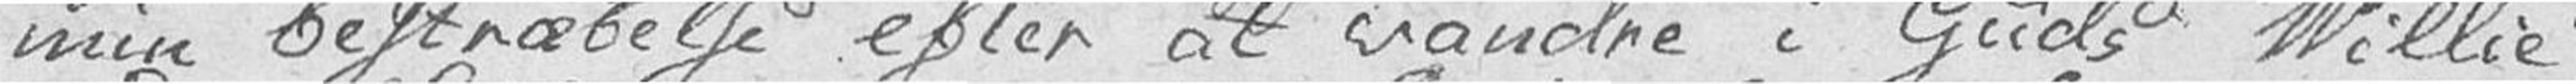min bestræbelse efter at vandre i Guds Villie
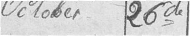October 26de
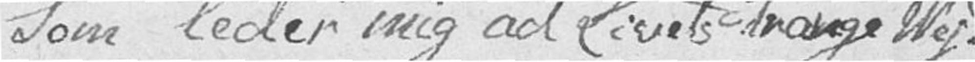Som leder mig ad Livets trange Vej.
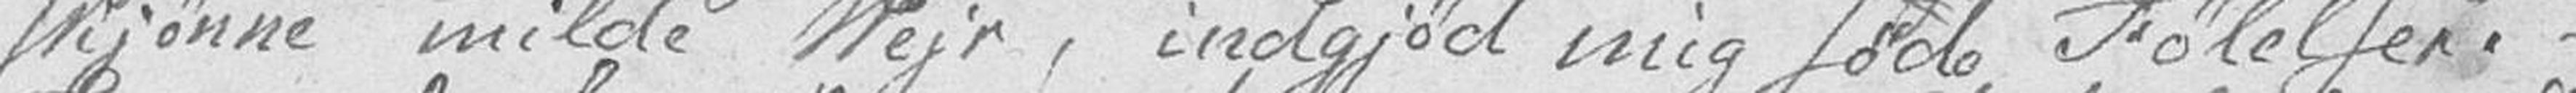skjønne milde Vejr, indgjød mig søde Følelser. –
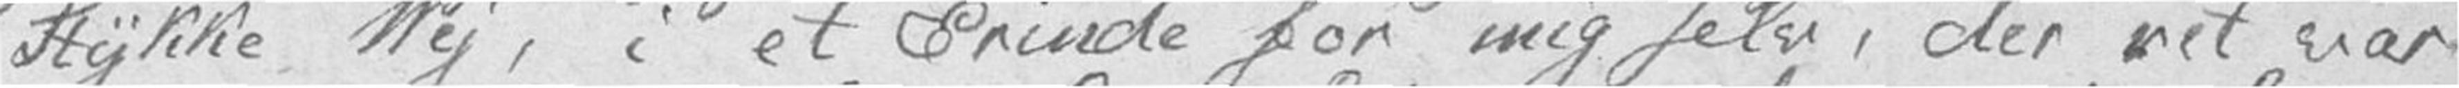Stykke Vej, i et Erinde for mig selv, der ret var
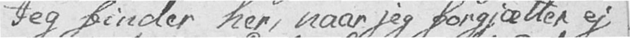Jeg finder her, naar jeg forgjætter ej
